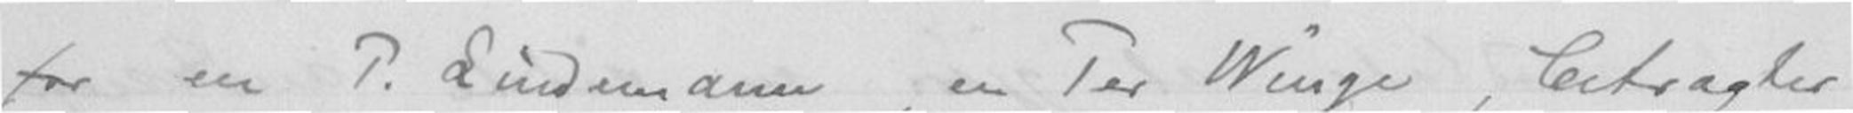for en P. Lindemann, en Per Winge, betragter
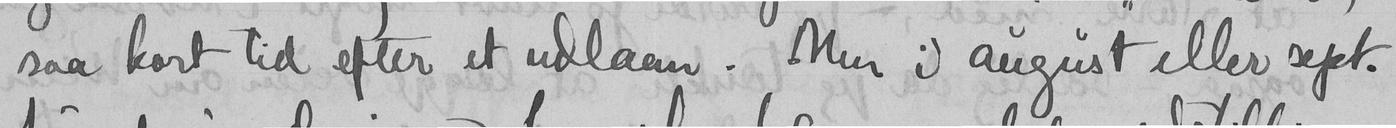saa kort tid efter et udlaan. Men i august eller sept.
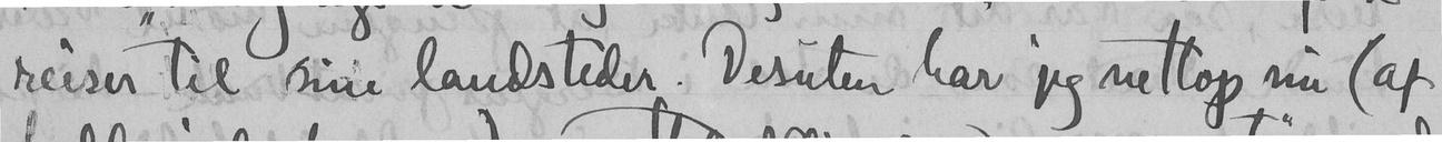reiser til sine landsteder. Desuten har jeg nettop nu (af
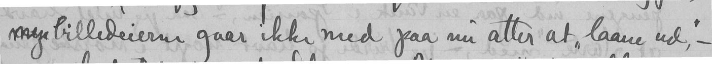men billedeierne gaar ikke med paa nu atter at "laane ud", -
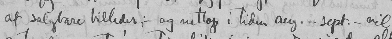af salgbare billeder; - og nettop i tiden aug. - sept. - vil
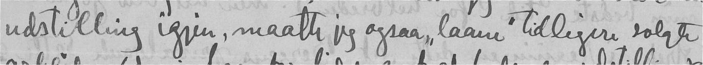udstilling igjen, maatte jeg ogsaa "laane" tidligere solgte
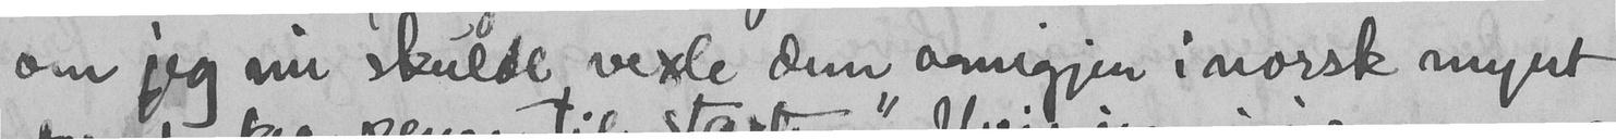om jeg nu skulde vexle dem omigjen i norsk mynt
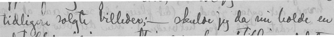tidligne solgte billeder; - skulde jeg da nu holde en
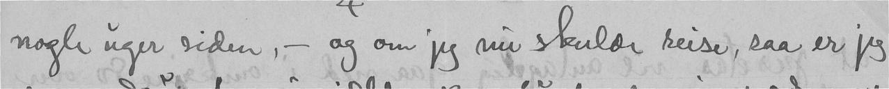nogle uger siden, - og om jeg nu skulde reise, saa er jeg
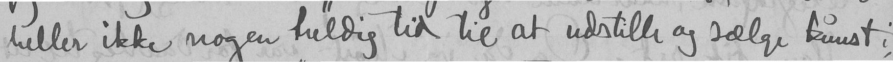heller ikke nogen heldig tid til at udstille og sælge kunst;
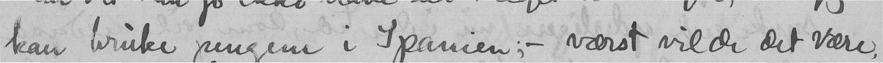kan bruke pengene i Spanien; - værst vilde det være,
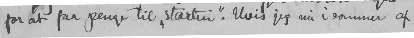for at faa penge til "starten". Hvis jeg nu i sommer af
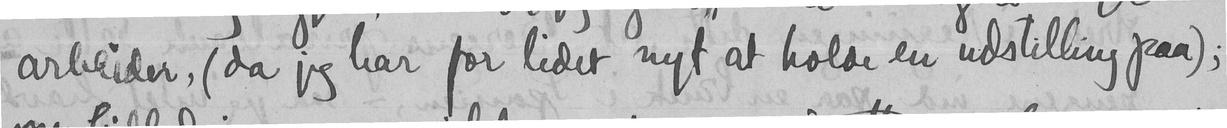arbeider, (da jeg har for lidet nyt at holde en udstilling paa);
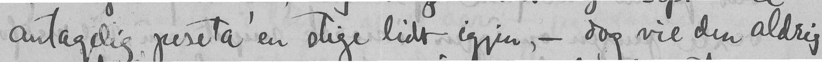antagelig peseta'en stige lidt igjen, - dog vil den aldrig
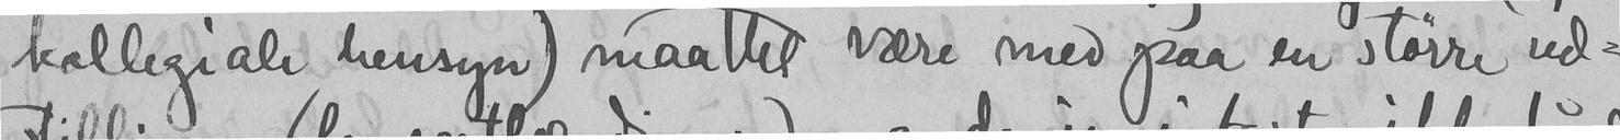kollegiale hensyn) maattet være med paa en større ud-
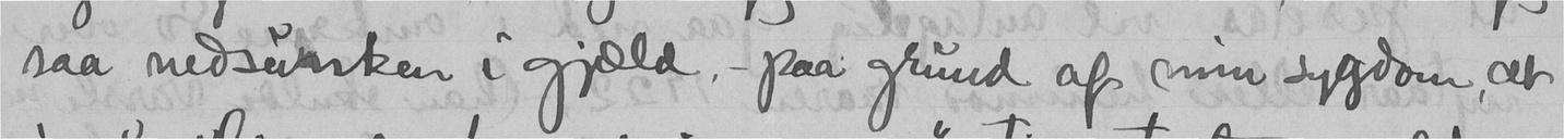saa nedsunken i gjæld, - paa grund af min sygdom, at
